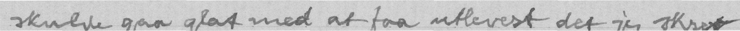skulde gaa glat med at faa utlevert det jeg skrev
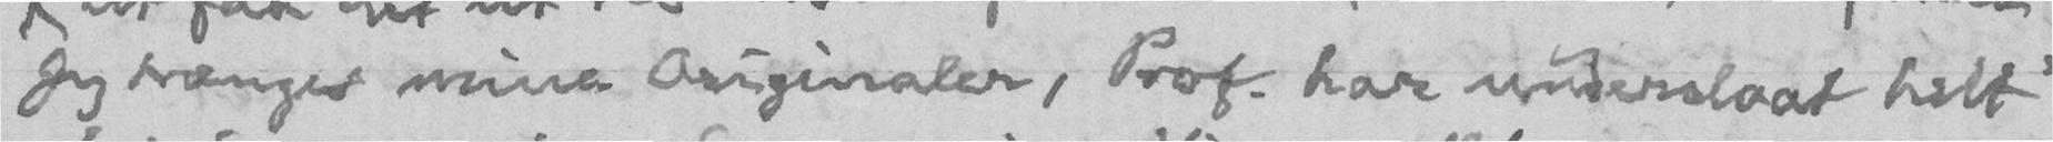Jeg trænger mine Originaler, Prof. har underslaat helt
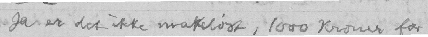Ja er det ikke makeløst, 1000 Kroner for
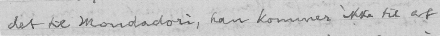det til Mondadori, han kommer ikke til at
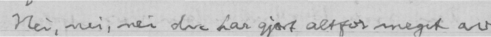Nei, nei, nei du har gjort altfor meget av
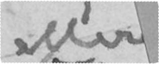eller
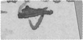og
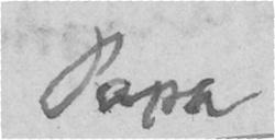Papa
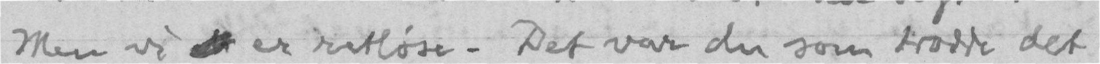Men vi  er retløse. Det var du som trodde det
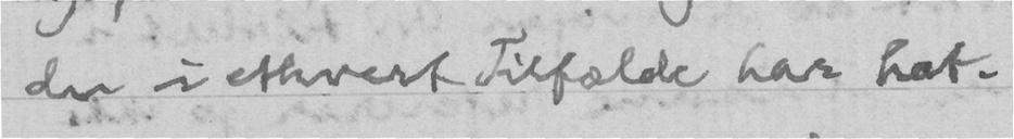du i ethvert Tilfælde har hat.
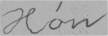Høn
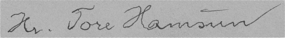Hr. Tore Hamsun
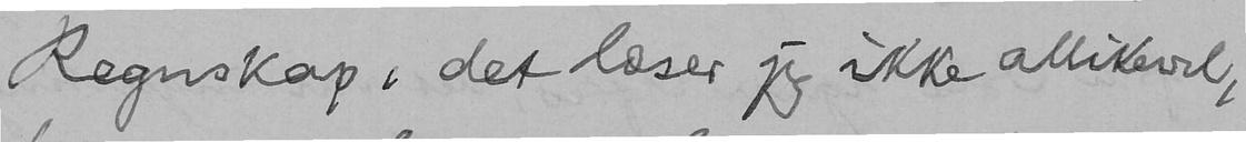Regnskap, det læser jeg ikke allikevel,
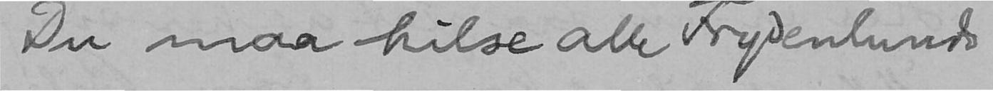Du maa hilse alle Frydenlunds
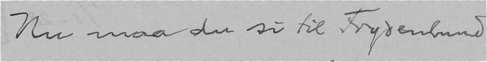Nu maa du si til Frydenlund
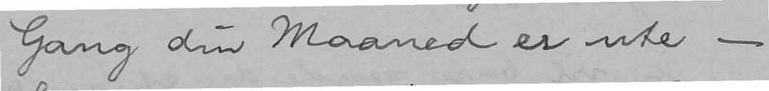Gang din Maaned er ute -
